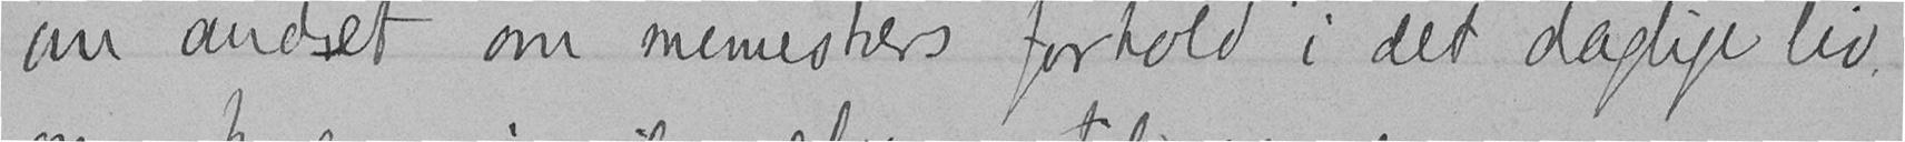om andet om menneskers forhold i det daglige liv,
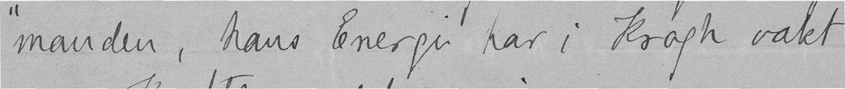manden, hans Energi har i Krogh vakt
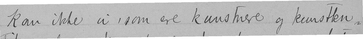Kan ikke vi, som ere kunstnere og kunstkri-
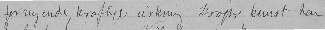fornyende kraftige virkning Kroghs kunst har
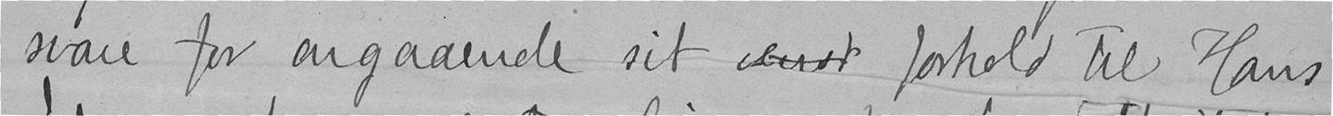svare for angaaende sit venst forhold til Hans
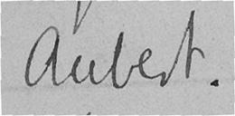Aubert.
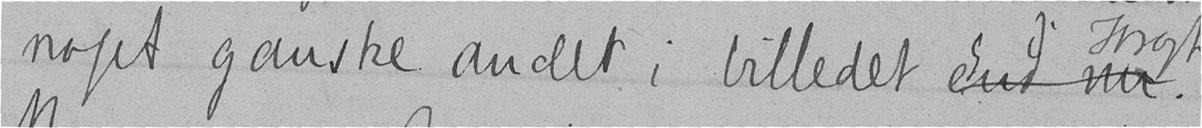noget ganske andet i billedet end nu.
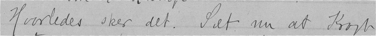Hvorledes sker det. Set nu at Krogh
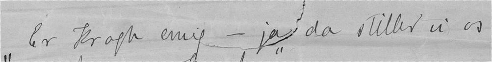"Er Krogh enig - ja da stiller vi os
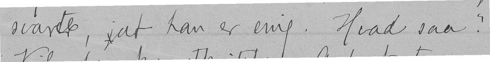svarte, jat han er enig. Hvad saa?
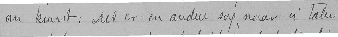om kunst. Det er en anden sag, naar vi taler
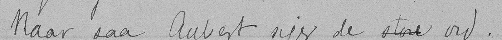Naar saa Aubert siger de store ord.
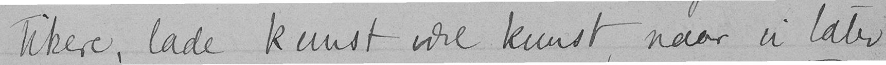tikere, lade kunst være kunst, naar vi taler
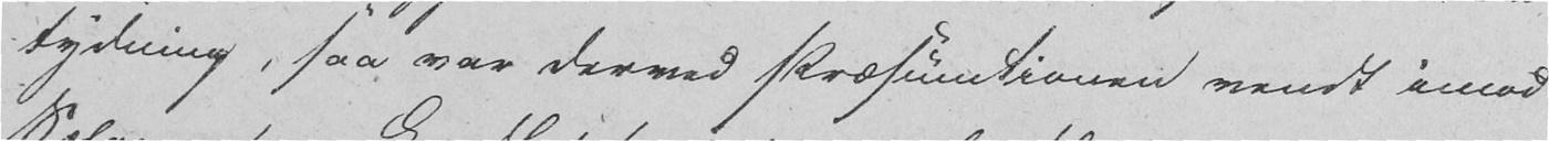tydning, saa var derved Præsumtionen vendt imod
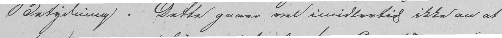Betydning. Dette gaaer vel imidlertid ikke an at
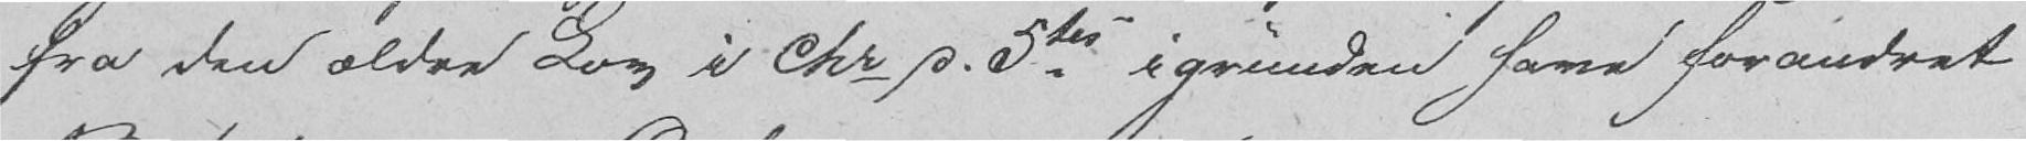fra den ældre Lov i Chr d. 5tes igrunden have forandret
